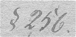§ 256.
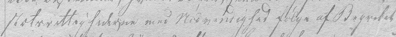stætsrettighederne med Nødvendighed følge af Begrebet
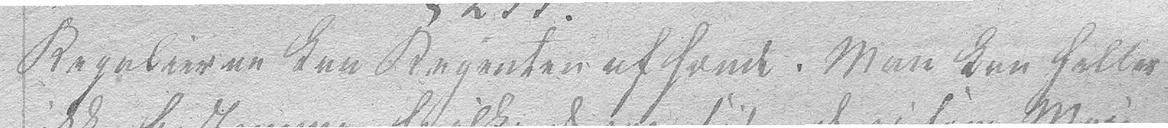Regalierne kan Regenten afhænde. Man kan heller
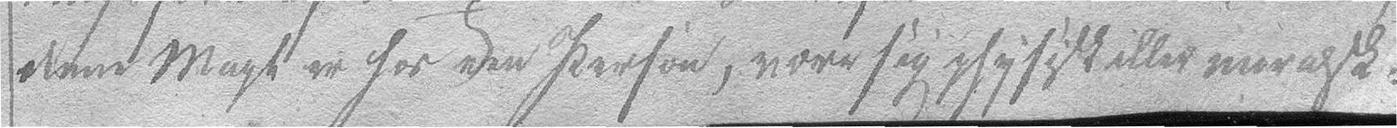denne Magt er hos een Person, være sig physisk eller moralsk.
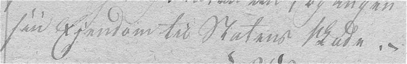sin Eiendom til Statens Skade. –
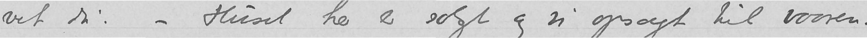ved du.  – Huset her er solgt og vi opsagt til vaaren.
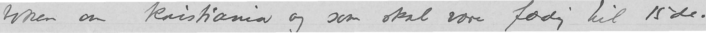boken om kristiania og som skal være færdig til 15de.
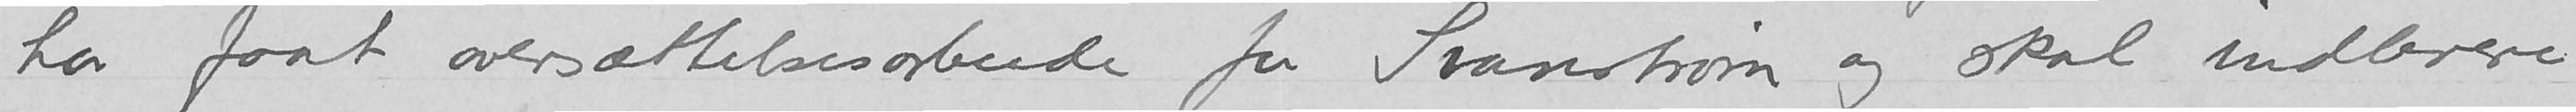har faat oversættelsesarbeide for Svanström og skal indlevere
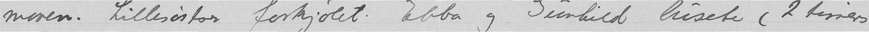maven. Lillesøster forkjølet. Ebba og Gunhild lusete (2 timers
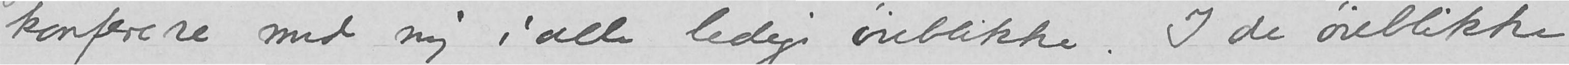konferere med mig i alle ledige øieblikke. I de øieblikke
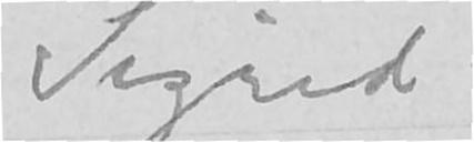Sigrid.
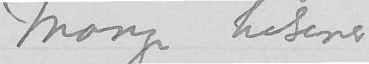Mange hilsener
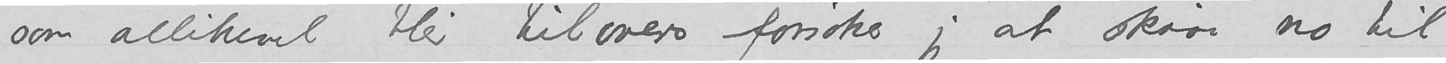som allikevel blir tilovers forsøker jeg at skrive no til
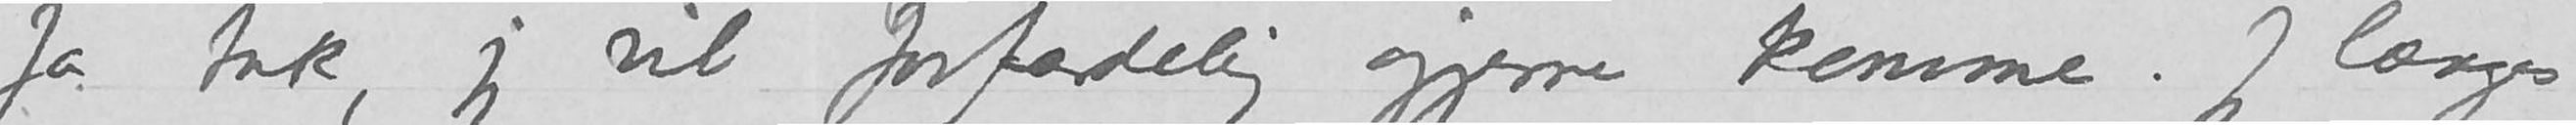Ja tak, jeg vil forfærdelig gjerne komme. Jeg længes
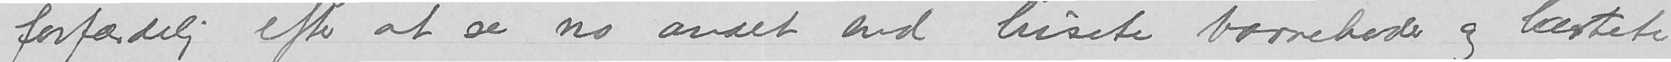forfærdelig efter at se no andet end lusete barnehoder og lastete
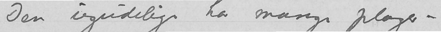Den ugudelige har mange plager –.
